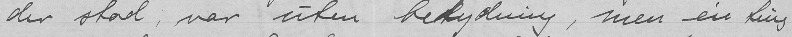der stod, var uten betydning, men en ting
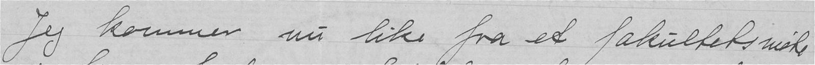Jeg kommer nu like fra et fakultetsmøte
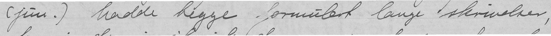(jun.) hadde begge formulert lange skrivelser,
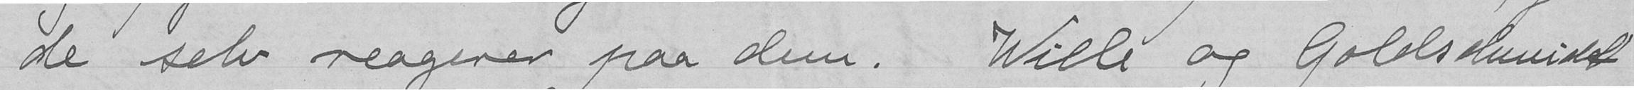de selv reagerer paa den. Wille og Goldschmidt
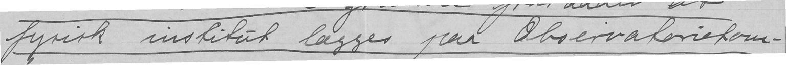fysisk institut lægges paa Observatorietom-
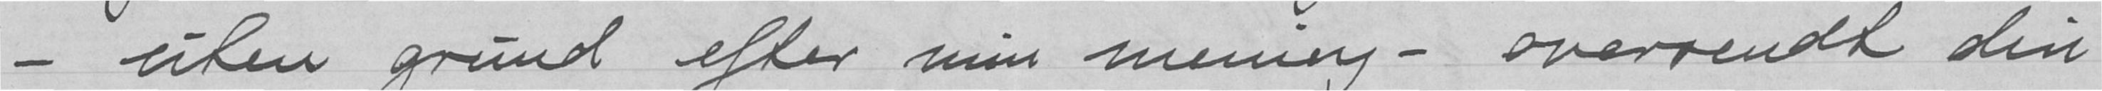- uten grund efter min mening - oversendt din
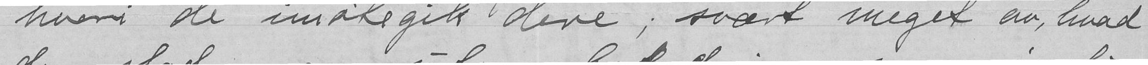hvori de imøtegik dere; svært meget av, hvad
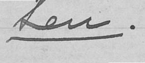ten.
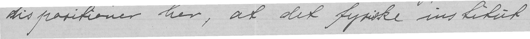dispositioner her, at det fysiske institut
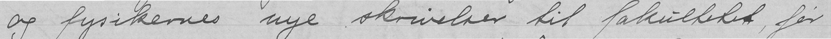og fysikernes nye skrivelser til fakultetet, før
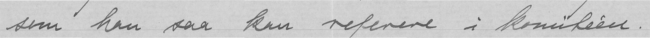som han saa kan referere i komiteén.
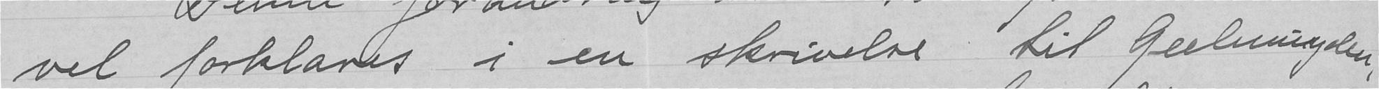vel forklares i en skrivelse til Geelmugden,
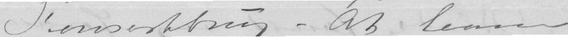Konsertbrug. At laane
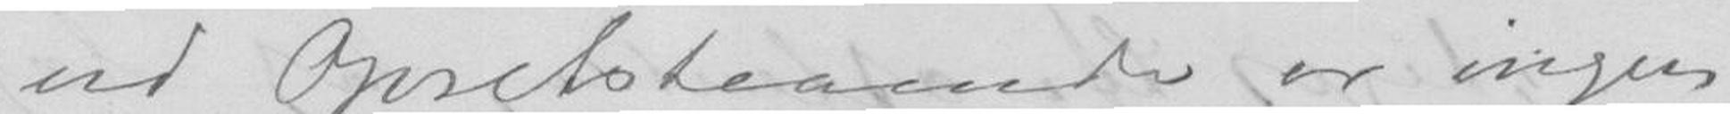ud Opretstaaende er ingen
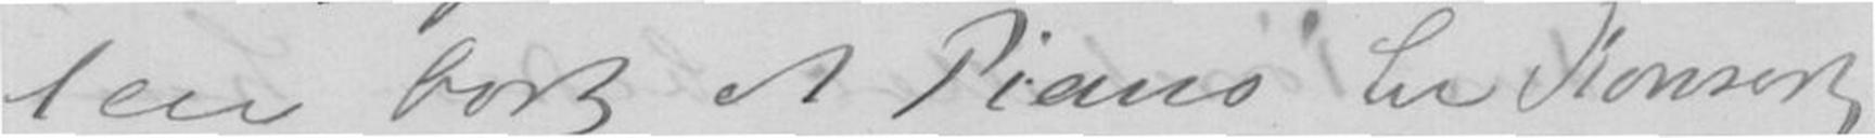leie bort et Piano til Konsert
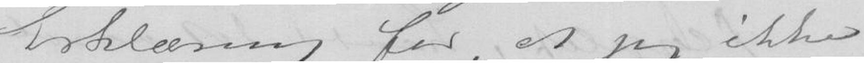Erklæring for, at jeg ikke
